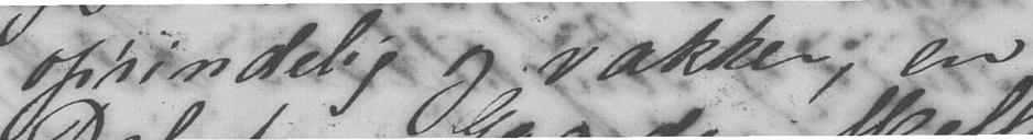oprindelig og vakker, en
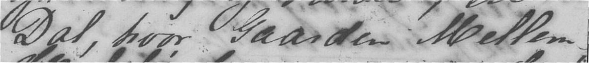Dal, hvor Gaarden Mellem-
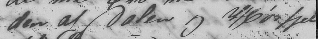den af Dalen og Høifjel
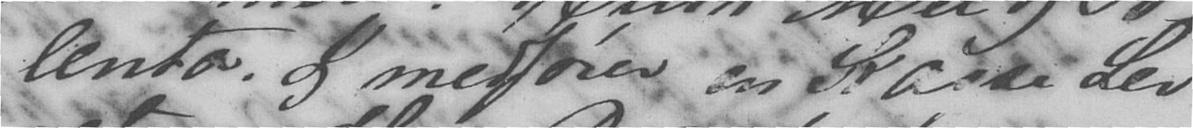lenta. Jeg medfører en Kasse Lev-
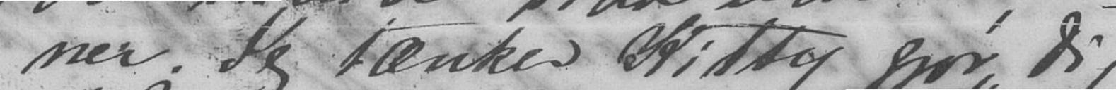ner. Jeg tænker Kitty gjør dig
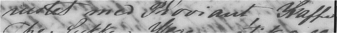rustet med Proviant, Kaffe,
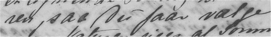ren, saa Du faar vælge
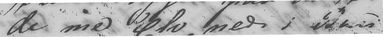de med Elv nede i Bun-
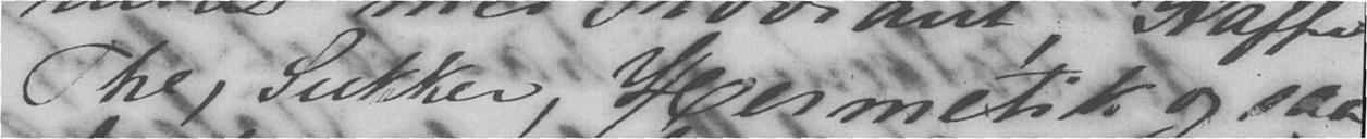The, Sukker, Hermetik og saa-
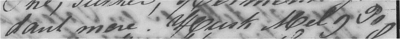dant mere! Husk Mel og Po-
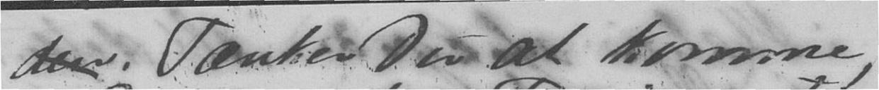den. Tænker Du at komme,
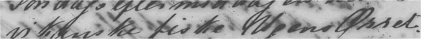vi kanske fiske Ugens Ørret.
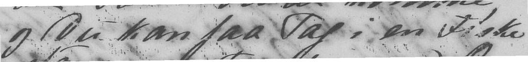og Du kan faa Tag i en Fiske
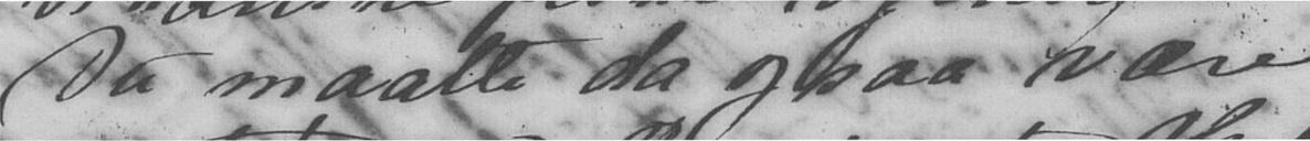Du maatte da ogsaa være
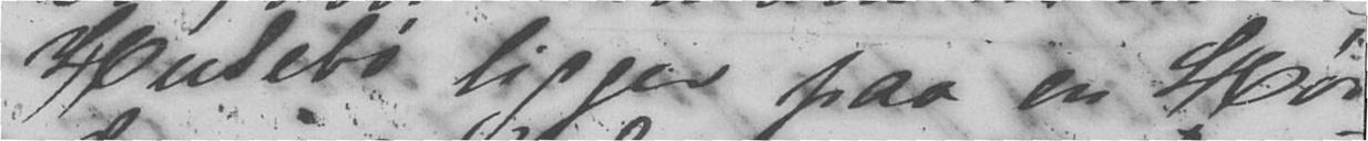Husebø ligger paa en Høi-
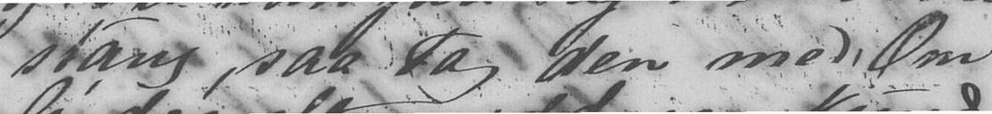stang, saa tag den med. Om
